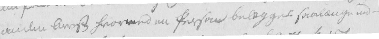anden Arrest hvorved en Persom belægges saalænge ind-
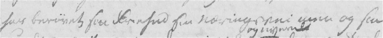har berøvet sin Friehed sin Næringsvei men og sin
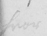hvor
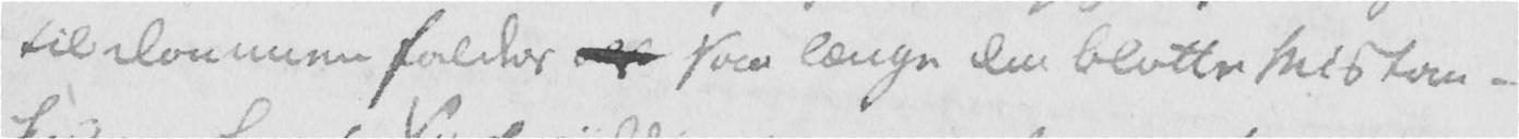til Dommen falder  saa længe den blotte Mistan-
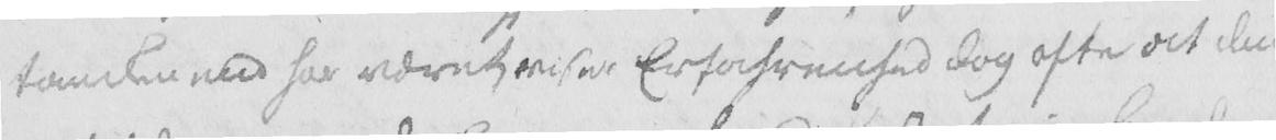tanken end har været viser Erfahrenhed dog ofte at den
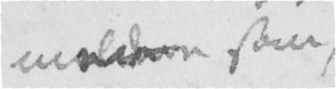mere som
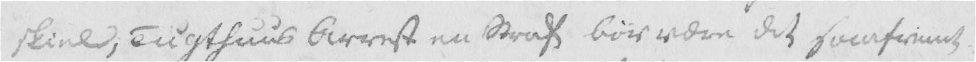skiel, Tugthuus Arrest er Straf bør være det saafremt
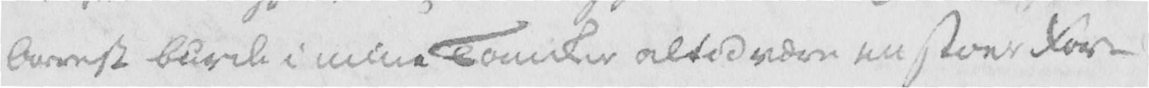Arrest burde i mine Tanker altid være en stoer For-
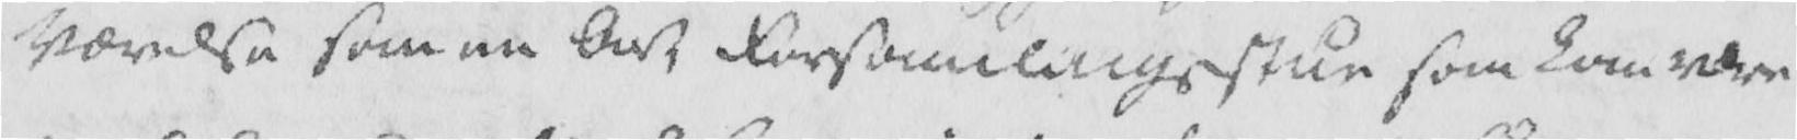Værelse som en Art Forsamlingsstue som kan være
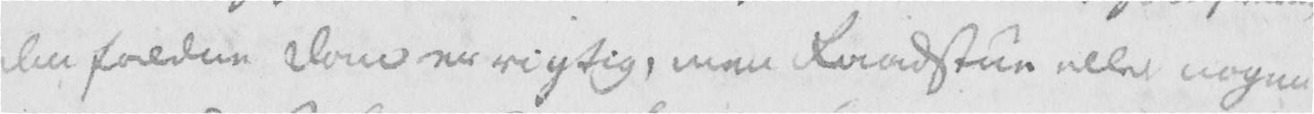den faldne Dom er rigtig, men Raadstue eller nogen
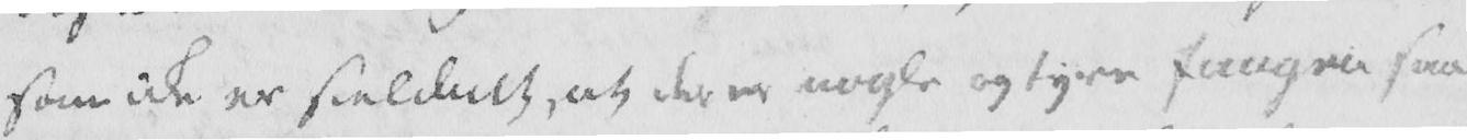som icke er sieldent, at der er nogle og tyve fanger saa
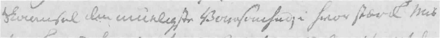Skaansel den mueligste Varsomhed; i hvor stærk Mis-
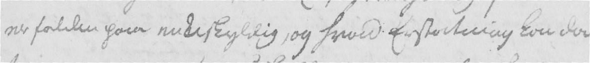er falden paa uskyldig, og hvad Erstatning kan da
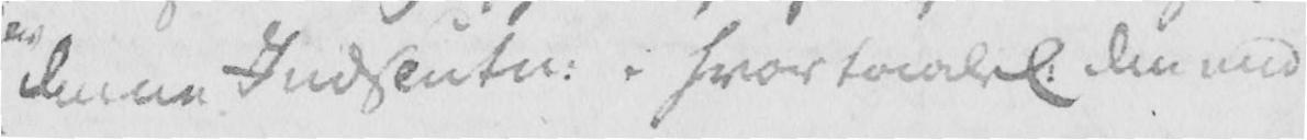denne Indslutn. i hvor taalel. den end
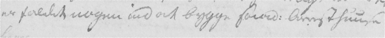er faldet nogen ind at bygge saad. Arresthuuse
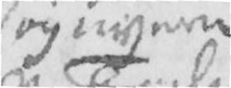og nyere
